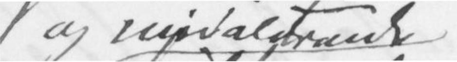og midaldrende
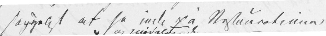sørgeligt at se inde på Restaurationer
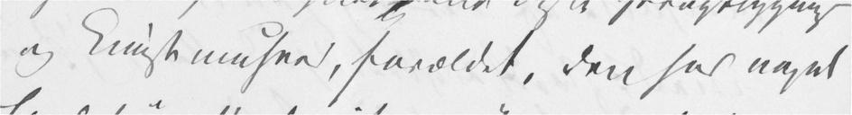og Kunstmuseer, forældet, den har noget
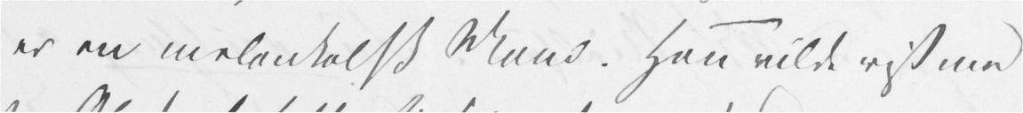er en melankolsk Mand. Han vilde vist med
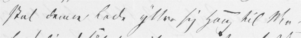skal denne Lede yttre sig Hang til Me-
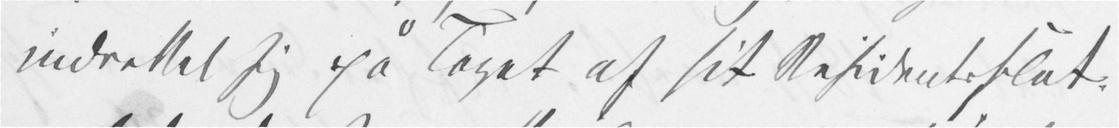indrettet sig på Taget af sit Residentsslot
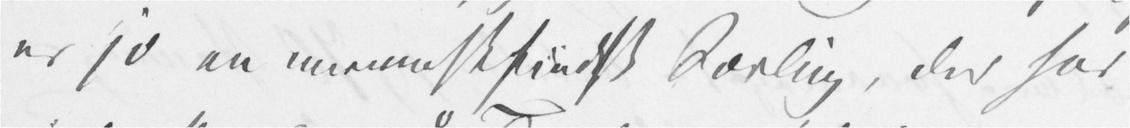er jo en menneskefiendsk Særling, der har
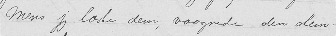Mens jeg læste dem, vaagnede den dem-
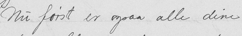Nu først er ogsaa alle dine
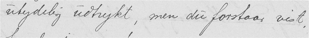utydelig udtrykt, men du forstaar vist,
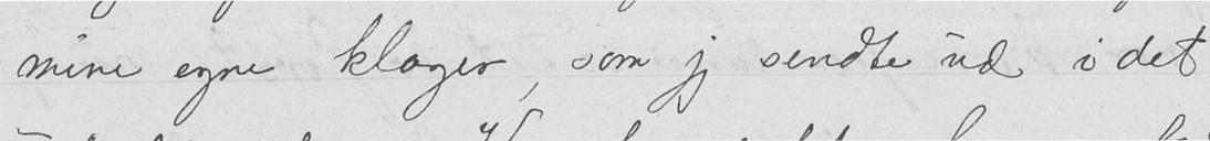mine egne klager, som jeg sendte ud i det
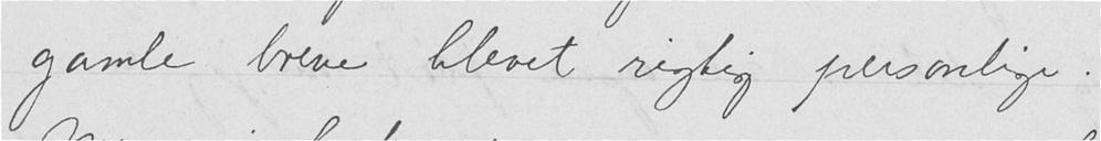gamle breve blevet rigtig personlige.
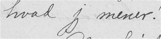hvad jeg mener!
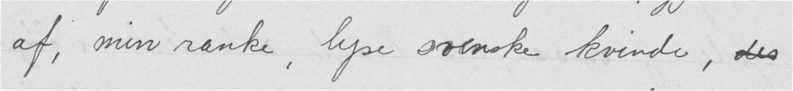af, min ranke, lyse svenske kvinde, der
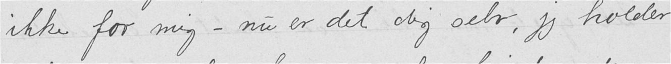ikke for mig – nu er det dig selv, jeg holder
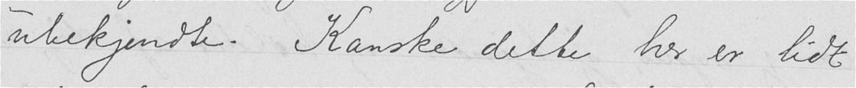ubekjendte. Kanske dette her er lidt
